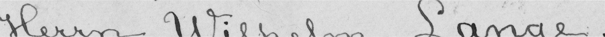Herrn Wilhelm Lange.
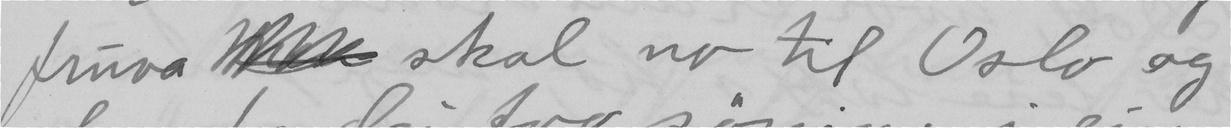fruva  skal no til Oslo og
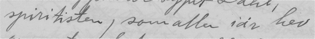spiritisten, som atter iår hev
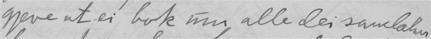gjeve ut ei bok um alle dei samtalar
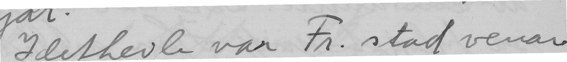Idetheile var Fr.stad venar
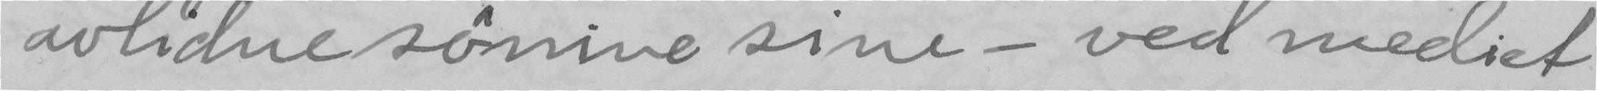avlidne sønine sine – ved mediet
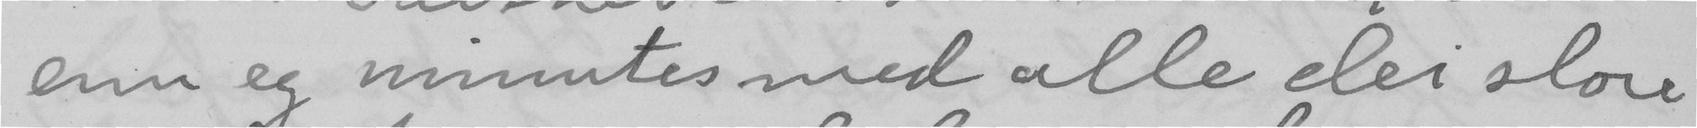enn eg minntes med alle dei store
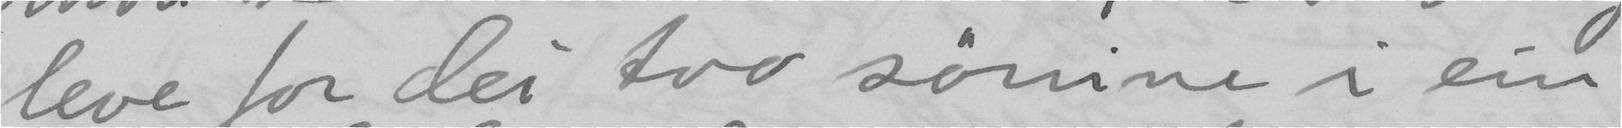leve for dei tvo söøninene i ein
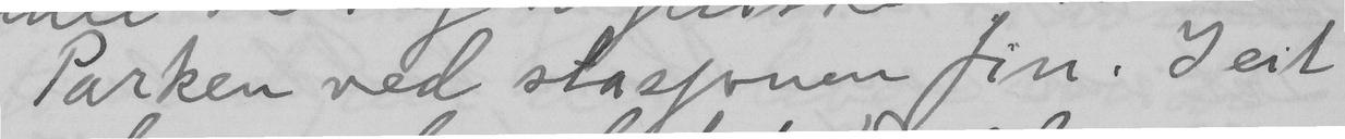Parken ved stasjonen fin. I eit
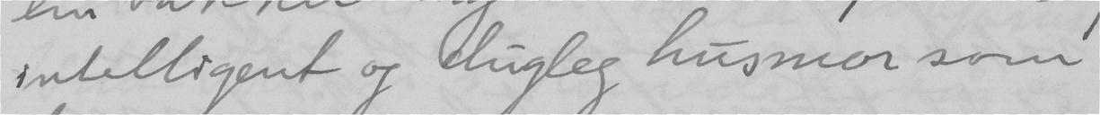intelligent og dugleg husmor som
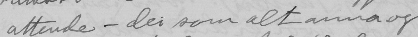attende – dei som alt anna og
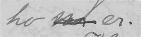ho  er.
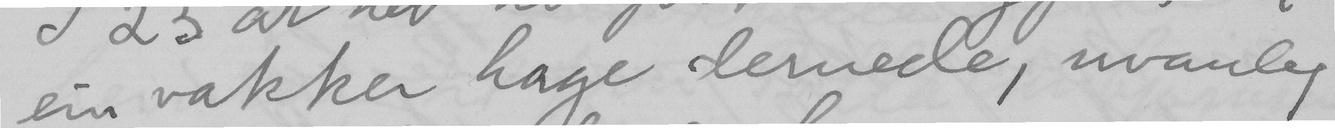ein vakker hage dernede, uvanleg
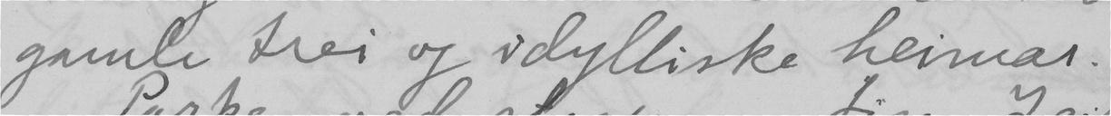gamle trei og idylliske heimar.
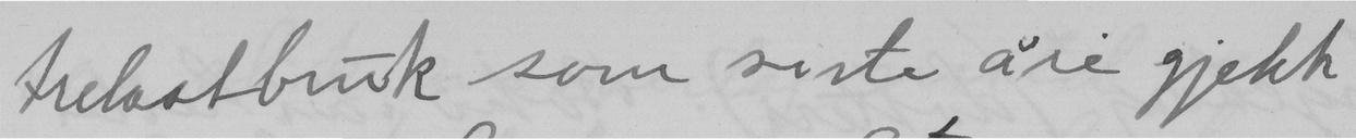trelastbruk som siste åre gjekk
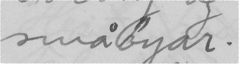småbyar.
